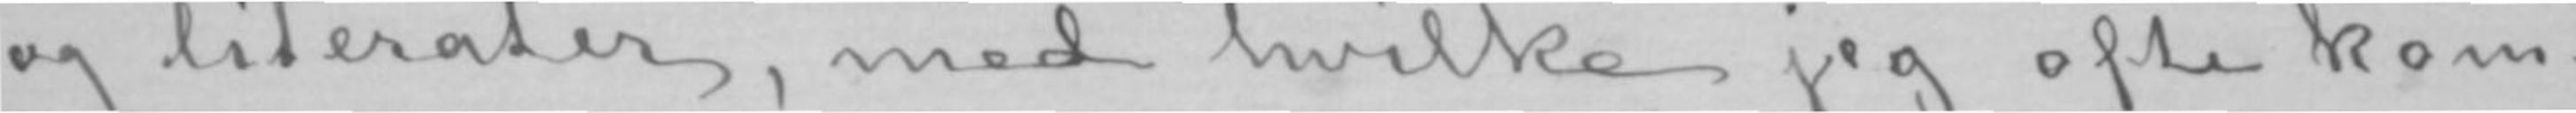og literater, med hvilke jeg ofte kom-
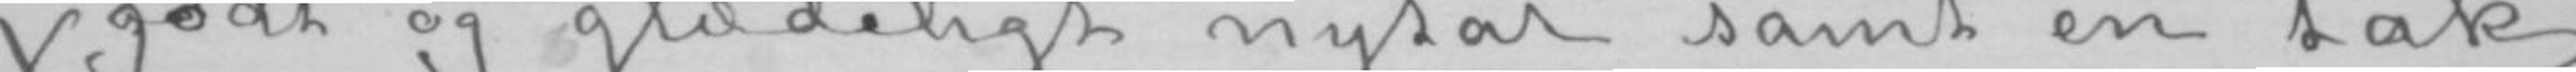godt og glædeligt nytår samt en tak
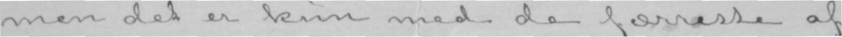men det er kun med de færreste af
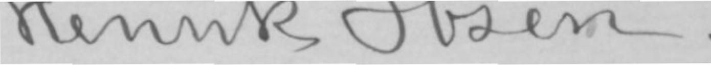Henrik Ibsen.
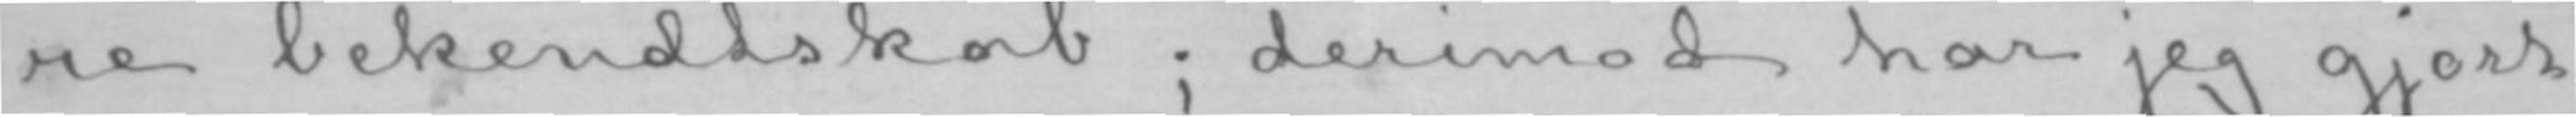re bekendtskab; derimod har jeg gjort
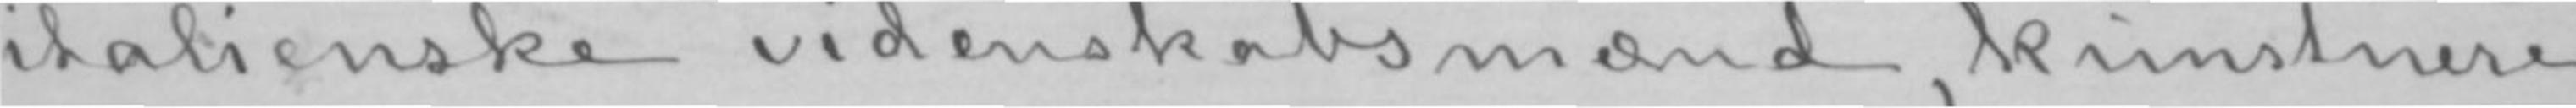italienske videnskabsmænd, kunstnere
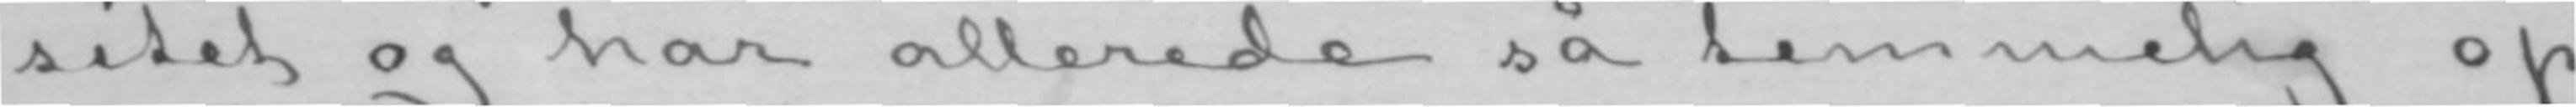sitet og har allerede så temmelig op-
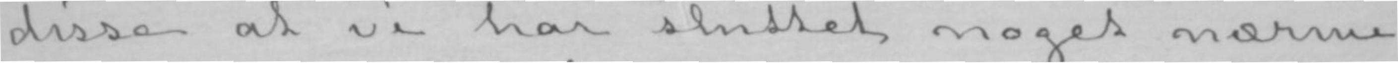disse at vi har sluttet noget nærme-
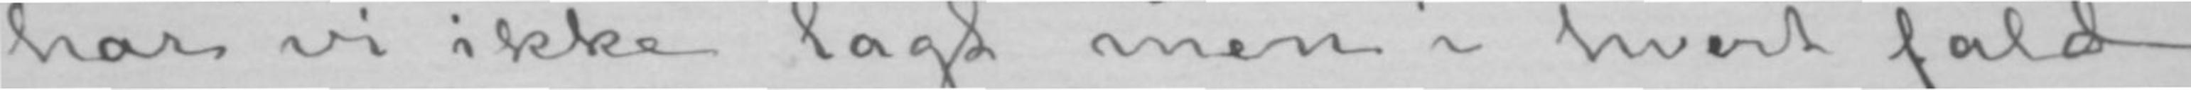har vi ikke lagt men i hvert fald
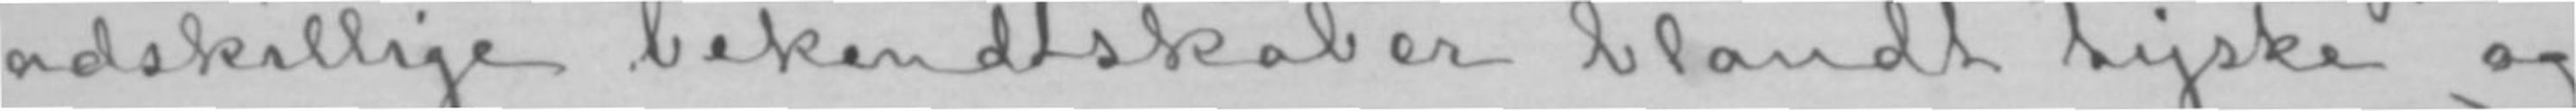adskillige bekendtskaber blandt tyske og
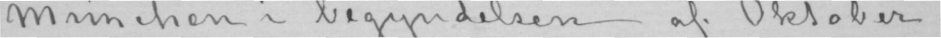München i begyndelsen af Oktober.
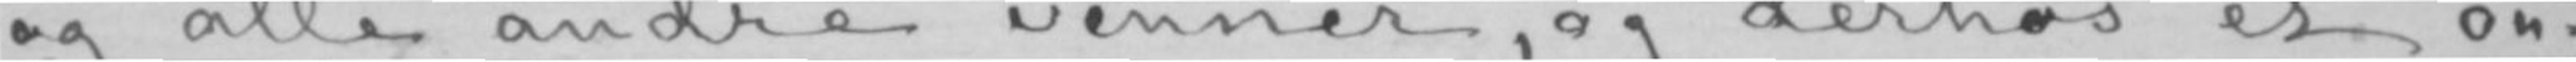og alle andre venner, og derhos et øn-
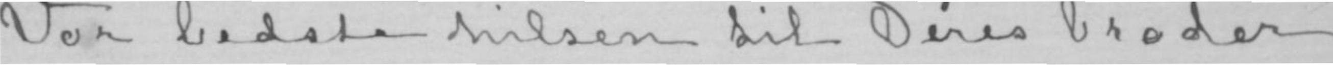Vor bedste hilsen til Deres broder
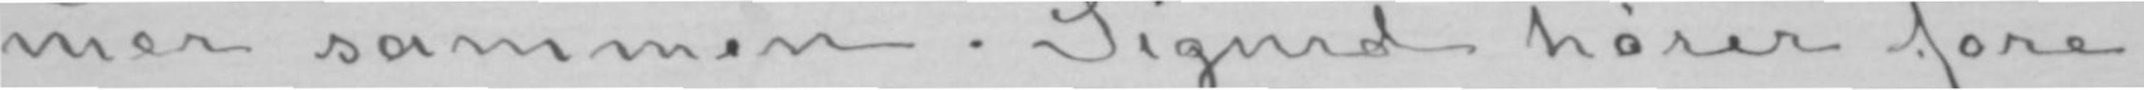mer sammen. Sigurd hører fore-
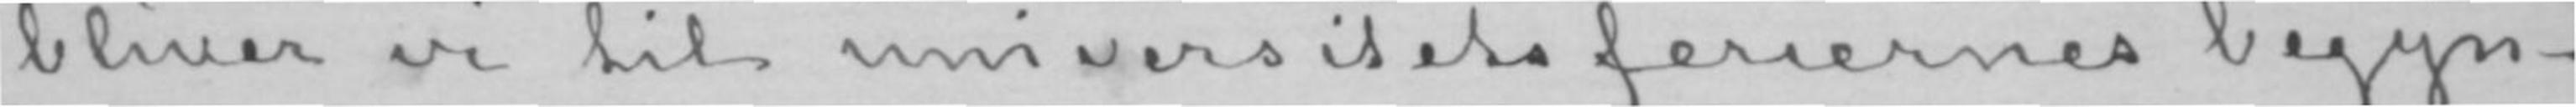bliver vi til universitetsferiernes begyn-
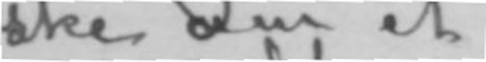ske om et
